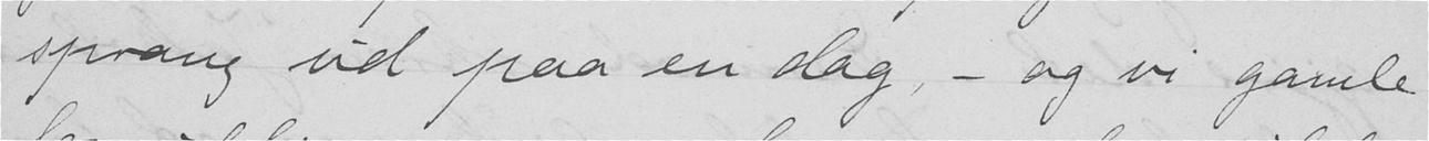sprang ud paa en dag, - og vi gamle
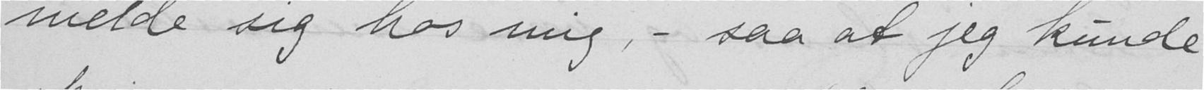melde sig hos mig,- saa at jeg kunde
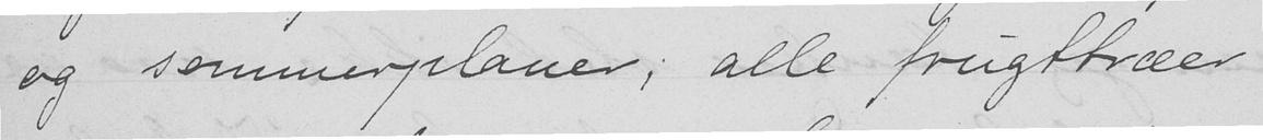og sommerplaner; alle frugttræer
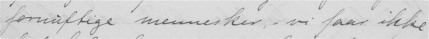fornuftige mennesker, - vi faar ikke
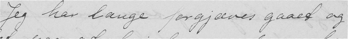Jeg har længe forgjæves gaaet og
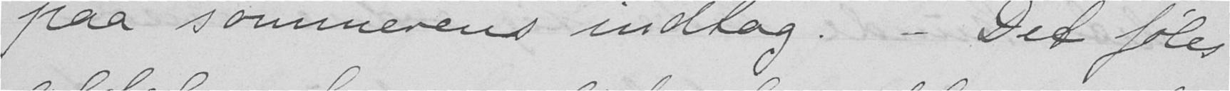paa sommerens indtog. - Det føles
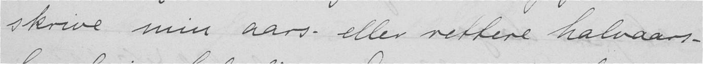skrive min aars- eller rettere halvaars-
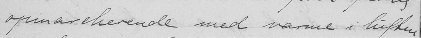opmarcherende med varme i luften
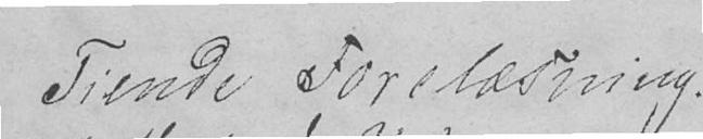Tiende Forelæsning.
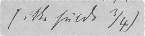(ikke fulde 3/4)
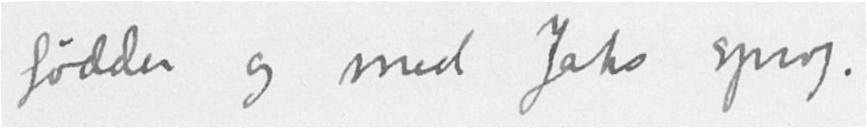fødder og med Jaks sprog.
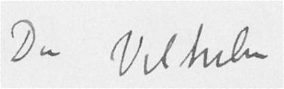Din Vilhelm
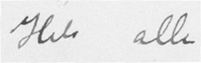Hils alle
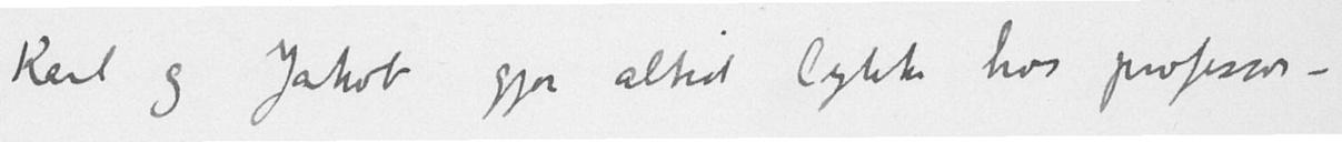Karl og Jakob gjør altid lykke hos professor-
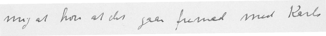mig at høre at det gaar fremad med Karls
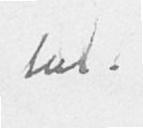tur.
